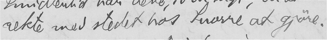rekte med stedet hos Snorre at gjøre.
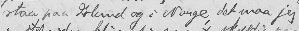staa paa Island og i Norge, det maa jeg
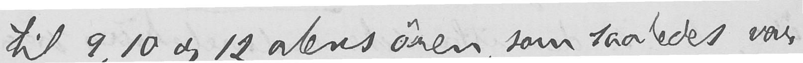til 9, 10 og 12 alens øren, som saaledes var
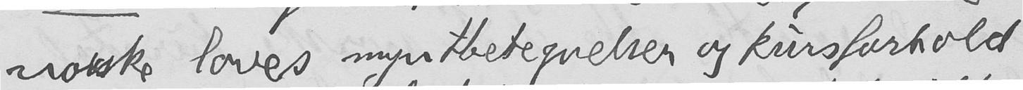norske loves myntbetegnelser og kursforhold
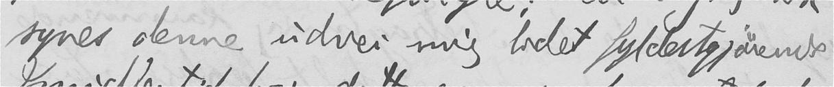synes denne udvei mig lidet fyldestgjørende.
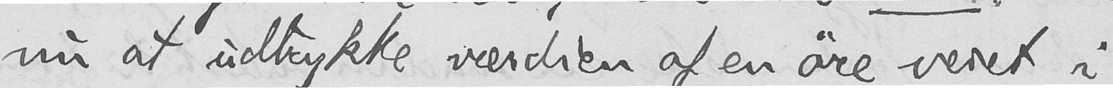nu at udtrykke værdien af en øre veiet i
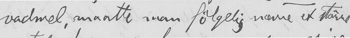vadmel, maatte man følgelig nævne et større
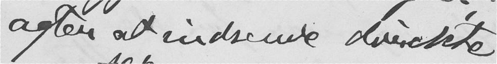agter at indsende direkte.
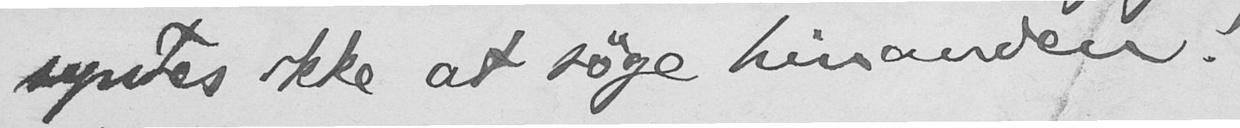syntes ikke at søge hinanden!
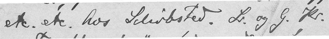etc. etc. hos Schibsted. L. og G. Kr.
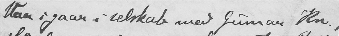Var i gaar i selskab med Gunnar Kr.
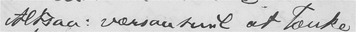Altsaa: værsaasnil at tænke
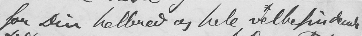for Din helbred og hele velbefindende
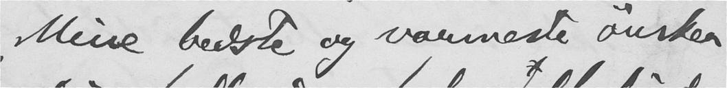Mine bedste og varmeste ønsker
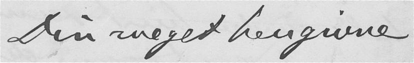Din meget hengivne
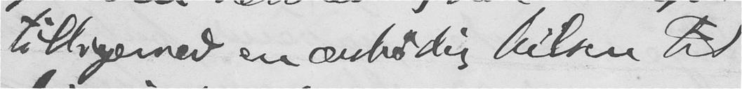tilligemed en ærbødig hilsen til
